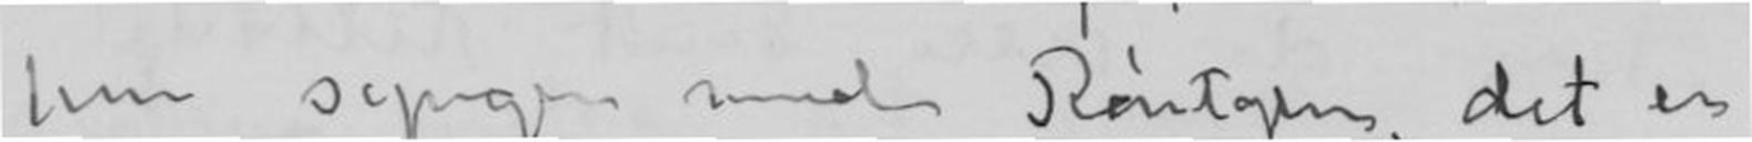hun synger med Röntgen, det er
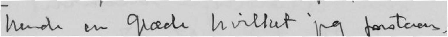hende en glæde hvilket jeg forstaar.
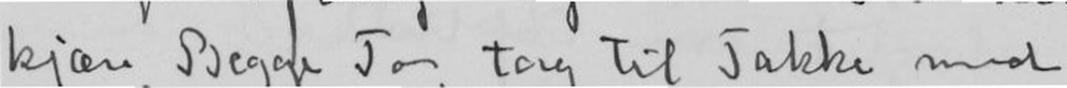kjære Begge To, tog til takke med
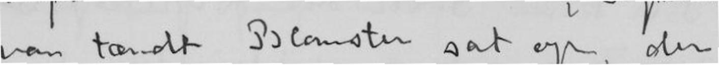var tændt Blomster sat op, der
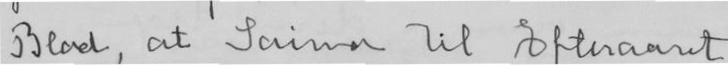Blad, at Saima til Efteraaret
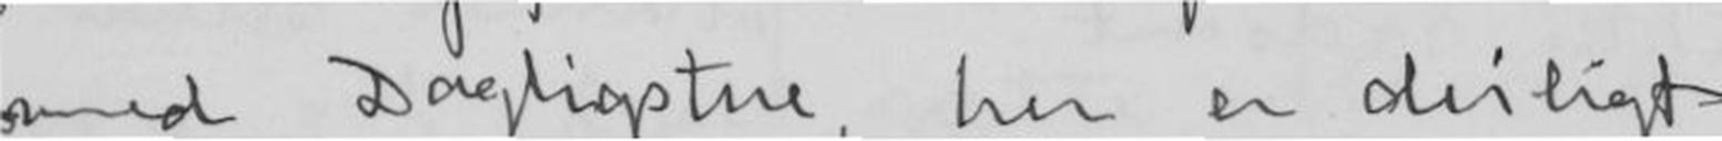med Dagligstue, her er deiligt
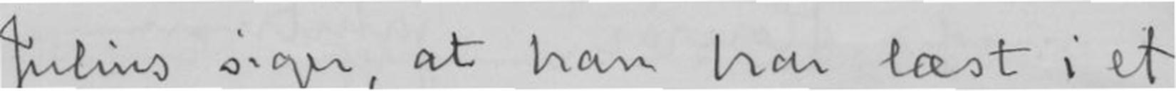Julius siger, at han har læst i et
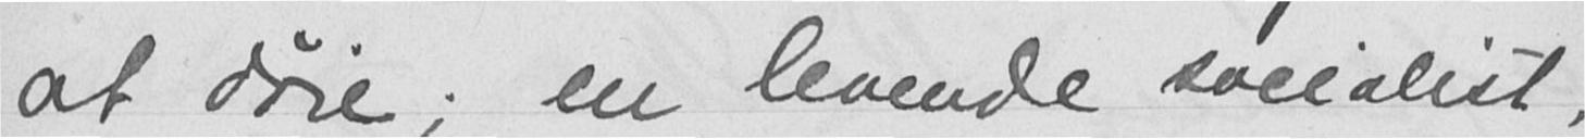at døie; en levende socialist,
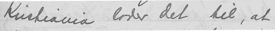Kristiania lader det til, at
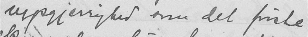nysgjerrighed som det første
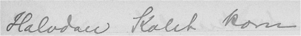Halvdan Koht kom
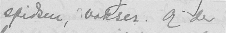spidsen, vokser. Og der
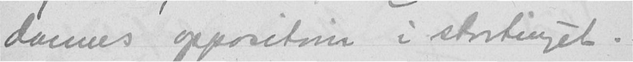dannes opposition i stortinget.
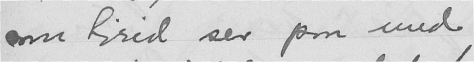som Sigrid ser paa med
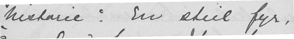historie". En steil fyr,
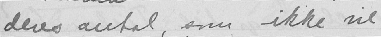deres antal, som ikke vil
